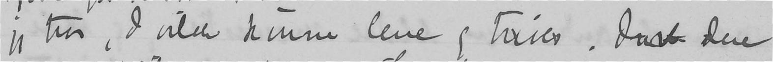jeg tror, I vilde kunne leve og trives. Just dere
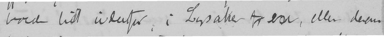boede lidt udenfor, i Lysaker fr ex, eller derom
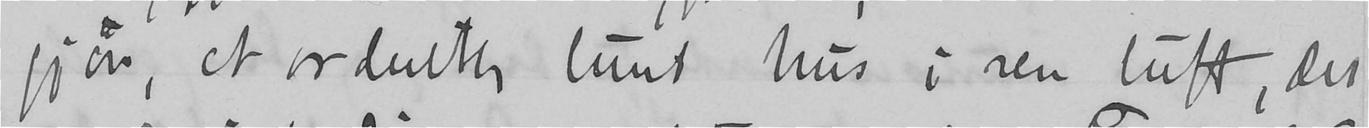gjør, et ordentlig lunt hus i ren luft, det
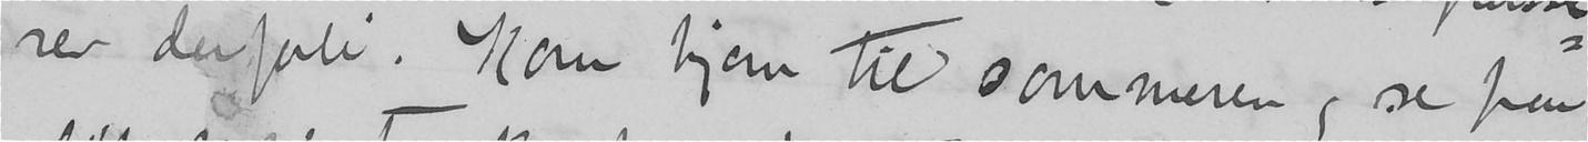rer der forbi. Kom hjem til sommeren og se paa
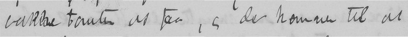vakkre tomter at faa, og der kommer til at
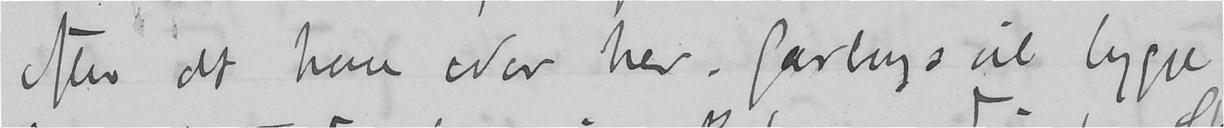efter at have eder her. Garborgs vil bygge
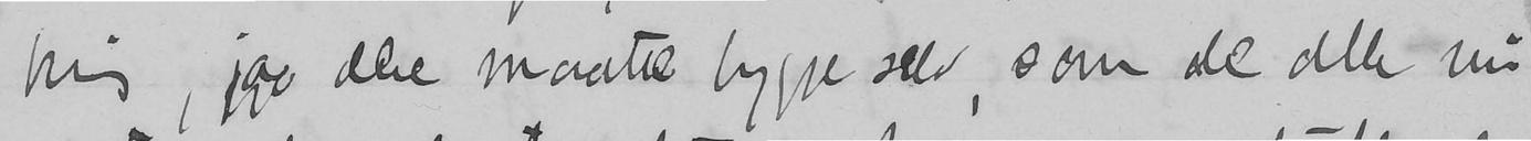kring, ja dere maatte bygge selv, som de alle nu
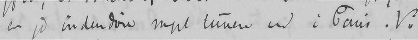er jo indendørs meget lunere end i Paris. Vi
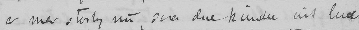er mer storby nu, saa dere kunde vist leve
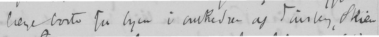længer borte fra byen i omkredsen af Tunsberg, Skien
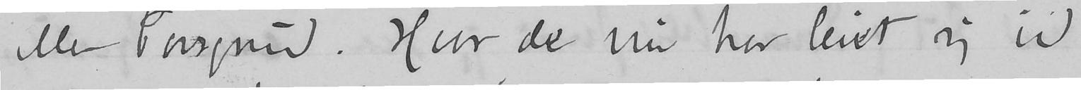eller Porsgrund. Hvor de nu har leiet sig ind
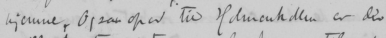hjemme. Ogsaa opad til Holmenkollen er der
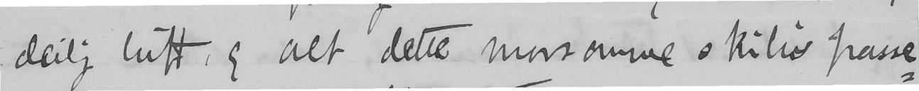deilig luft, og alt dette morsomme skiliv passe-
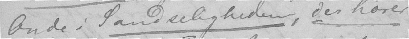Onde i Sandseligheden, der hører
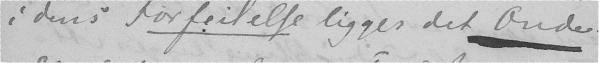i dens Forfeilelse ligger det Onde.
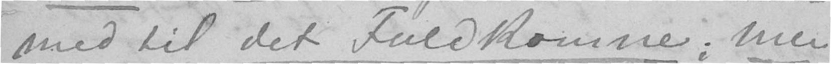med til det Fuldkomne; men
